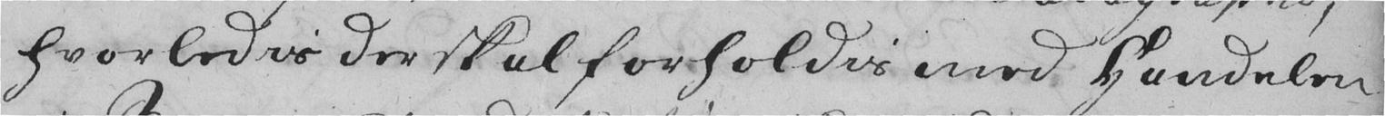hvorledis der skal forholdis med Handelen
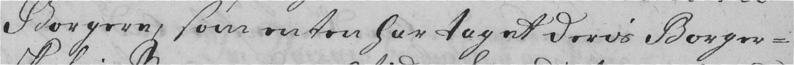Borgere, som enten har taget deres Borger-
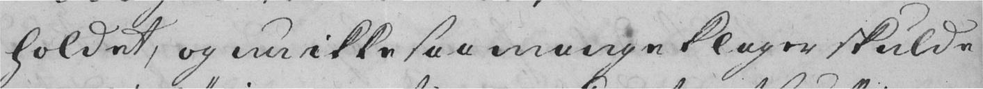holdet, og nu ikke saa mange klager skulde
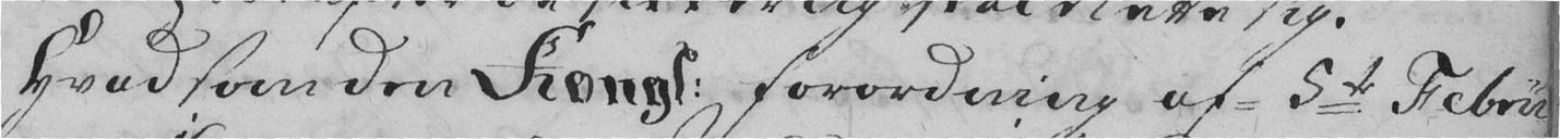Hvad som den Kongl. Forordning af 5te Febru-
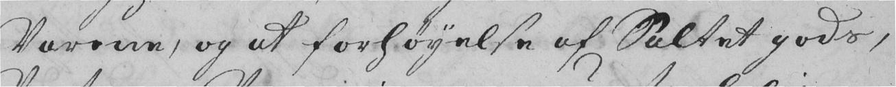Varene, og at forhøyelse af Saltet gods,
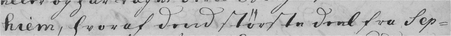hiem, hvoraf dend største deel fra Sep-
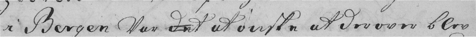i Bergen var  at ønske at derover blev
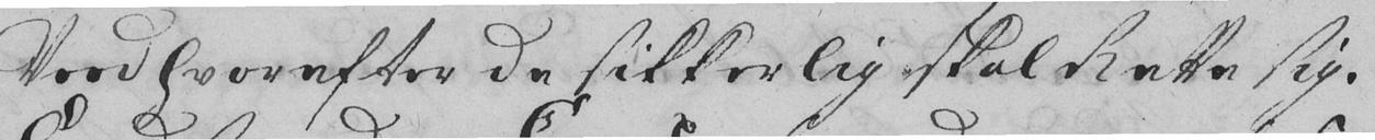Veed hvorefter de sikkerlig skal Rette sig.
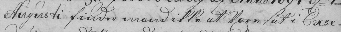Augusti finder mand ikke at Være sat i Exce-
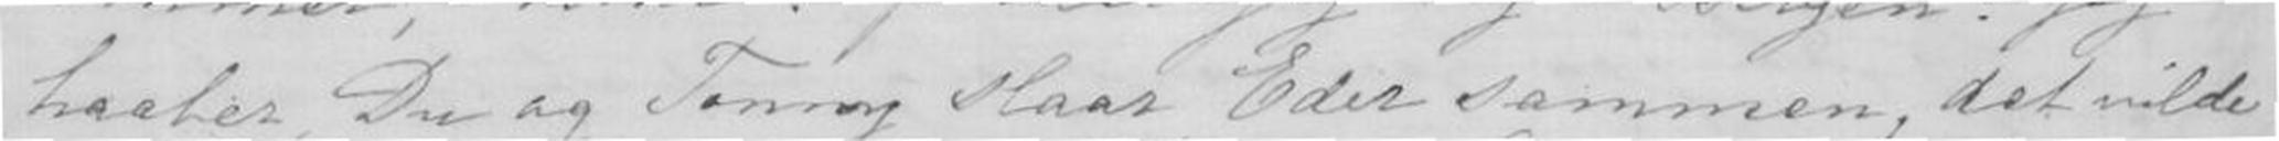haaber, Du og Tonny slaar Eder sammen, det vilde
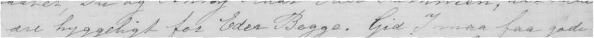ære hyggeligt for Eder Begge. Gid I maa faa gode
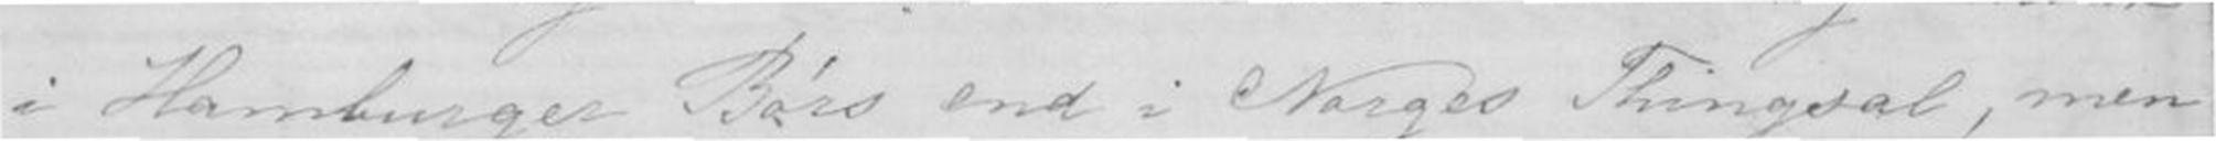i Hamburger Børs end i Norges Thingsal, men
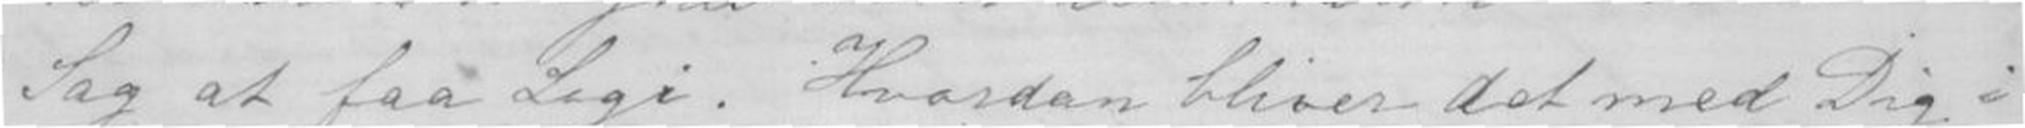Sag at faa Logi. Hvordan bliver det med Dig i
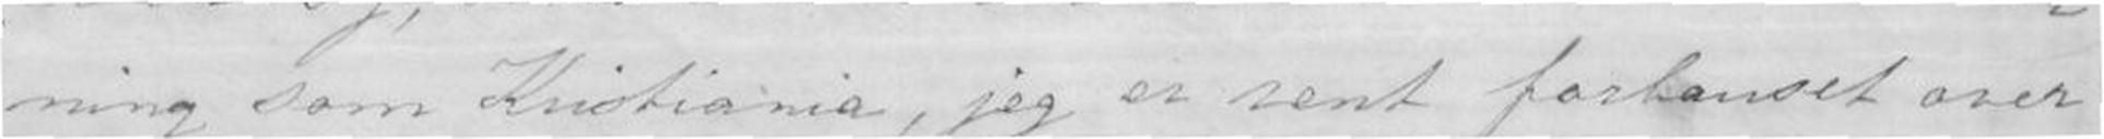ning som Kristiania, Jeg er rent forbauset over
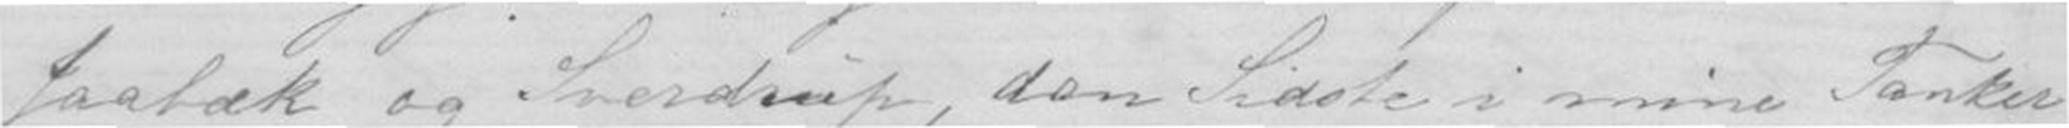Jaabæk og Sverdrup, den Sidste i mine Tanker
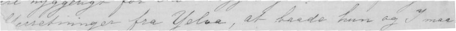erretninger fra Yelva, at baade hun og I maa
